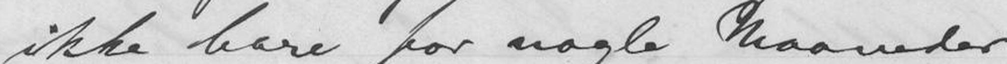ikke bare for nogle Maaneder
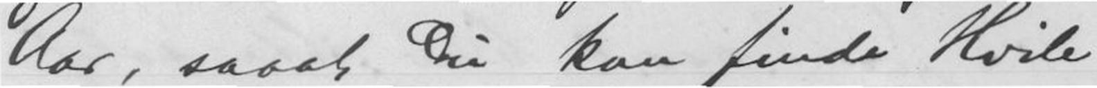Aar, saaat Du kan finde Hvile,
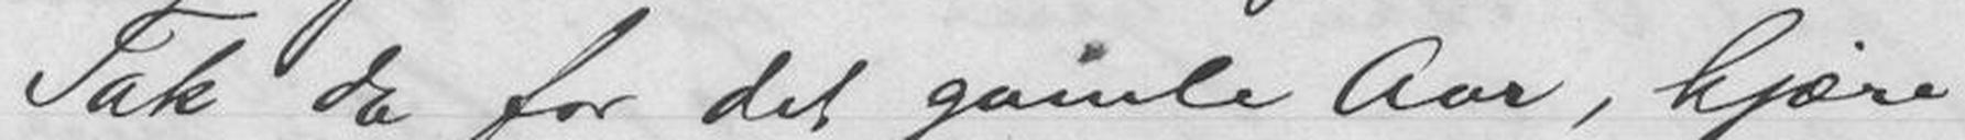Tak da for det gamle Aar, kjære
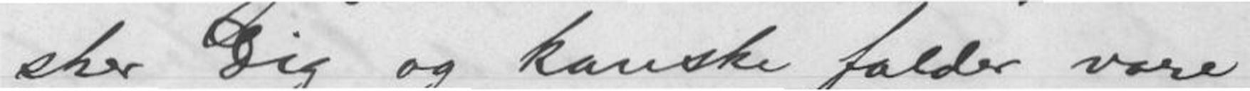sker Dig og kanske falder vore
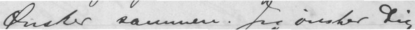Ønsker sammen. Jeg ønsker Dig
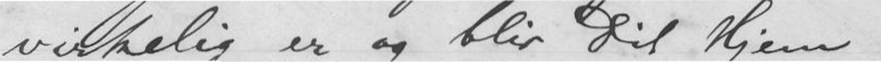virkelig er og blir Dit Hjem,
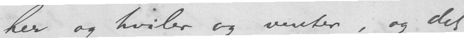her og hviler og venter, og det
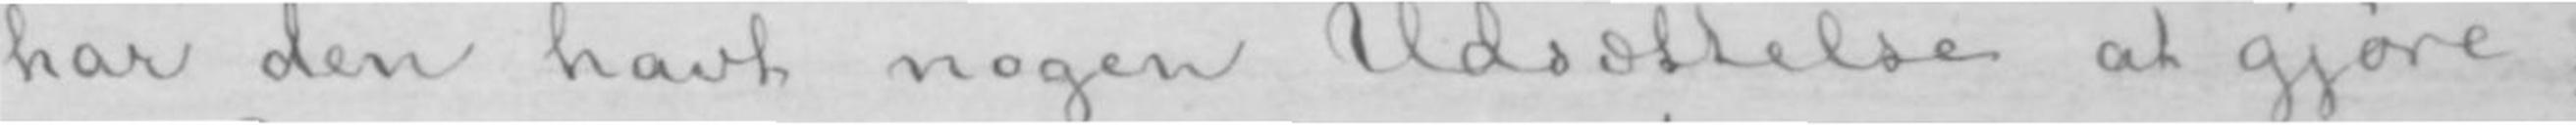har den havt nogen Udsættelse at gjøre,
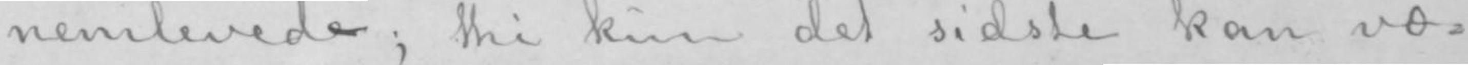nemlevede; thi kun det sidste kan væ-
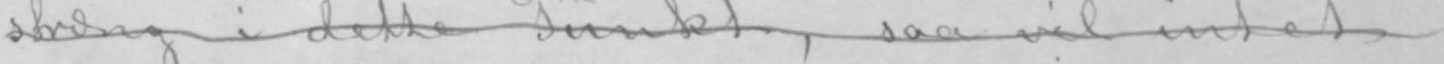stræng i dette Punkt, saa vil intet
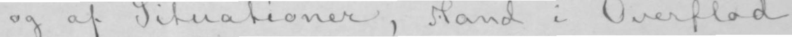og af Situationer, Aand i Overflod,
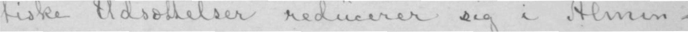tiske Udsættelser reducerer sig i Almin-
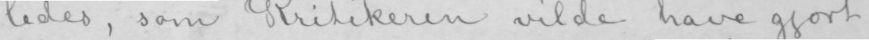ledes, som Kritikeren vilde have gjort-
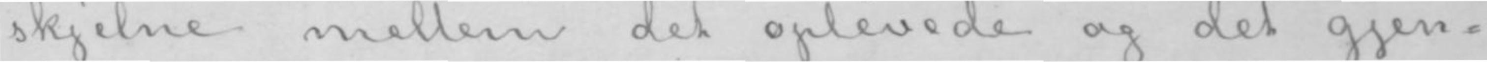skjelne mellem det oplevede og det gjen-
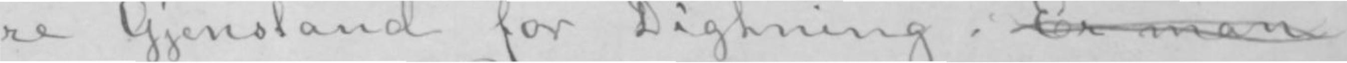re Gjenstand for Digtning. Er man
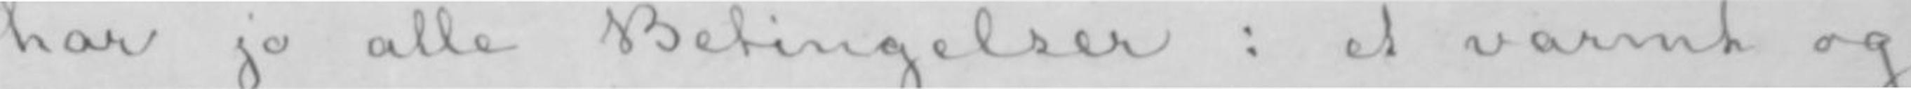har jo alle Betingelser: et varmt og
